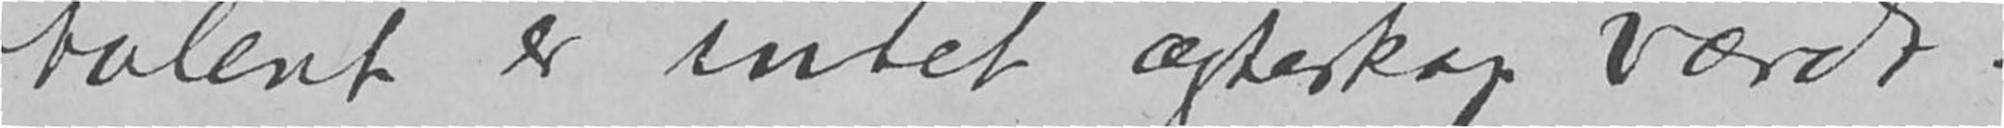talent er intet ægteskap værdt.
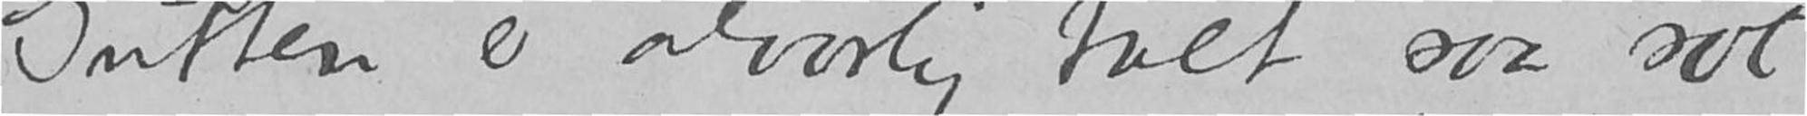Gutten er alvorlig talt saa søt
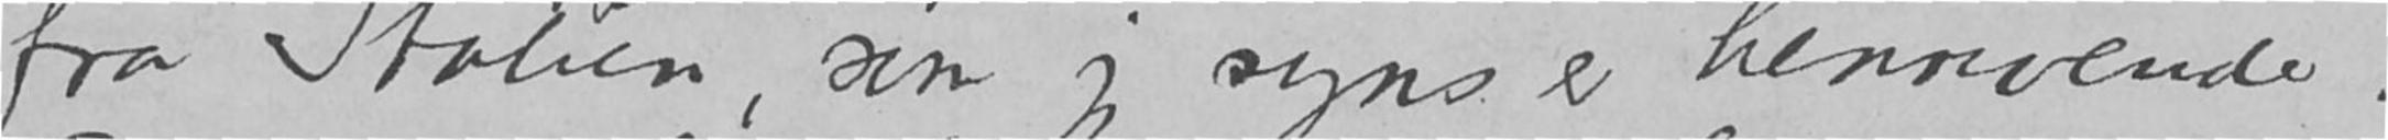fra Italien, som jeg synes er henrivende.
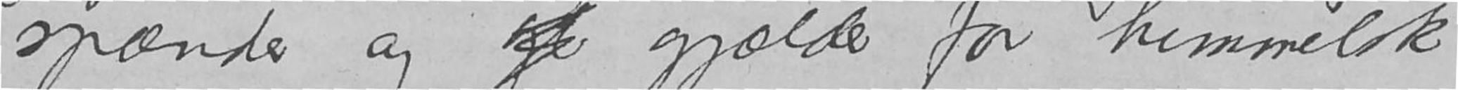spænder og  gjælder for himmelsk
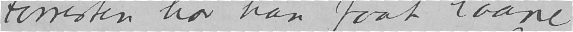Forresten har han faat laane
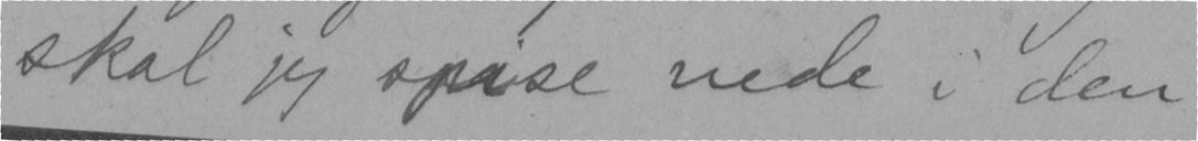skal jeg spise nede i den
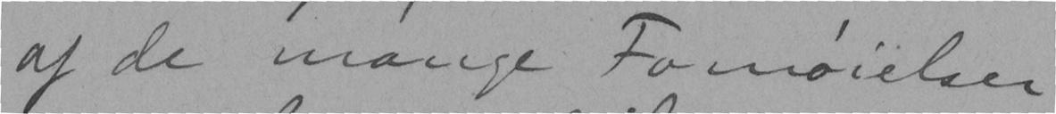af de mange Fornøielser
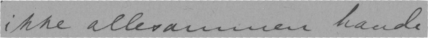ikke allesammen havde
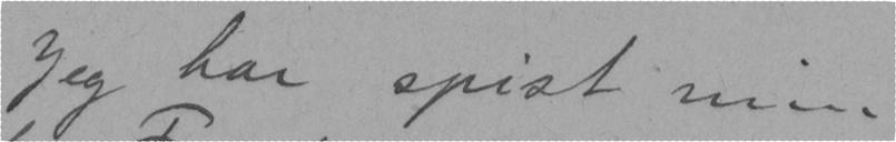Jeg har spist min
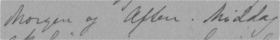Morgen og Aften. Middag
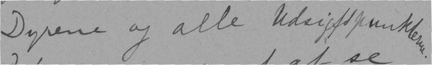Dyrene og alle Udsigtspunkterne.
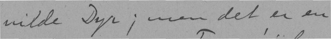vilde Dyr; men det er en
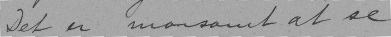Det er morsomt at se
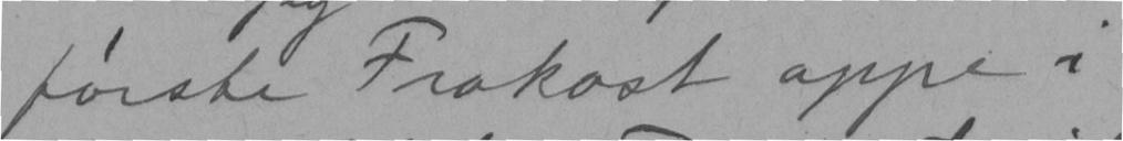første Frokost oppe i
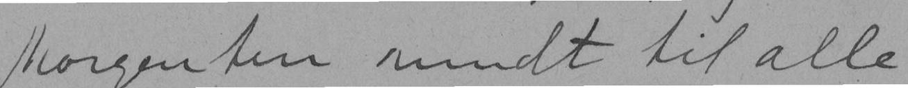morgentur rundt til alle
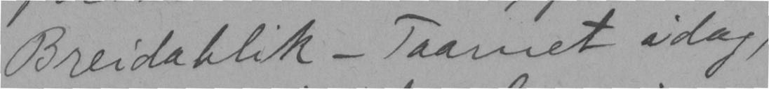Breidablik - Taarnet idag,
